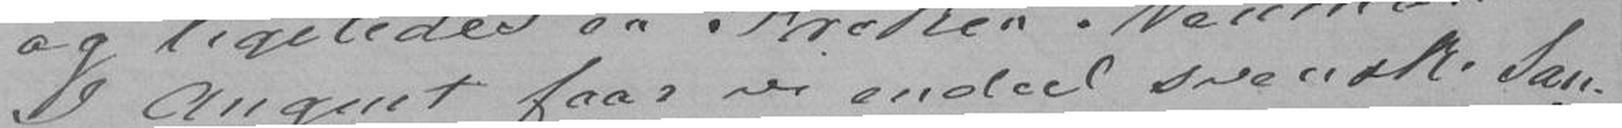I August faar vi endeel svenske San-
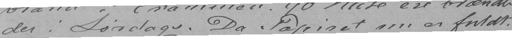der i Lørdags. Da Papiret nu er fuldt
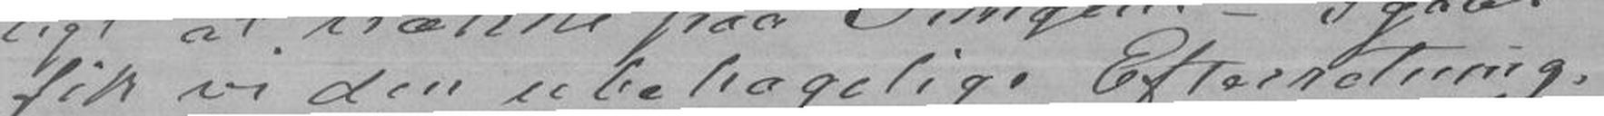fik vi den ubehagelige Efterretning
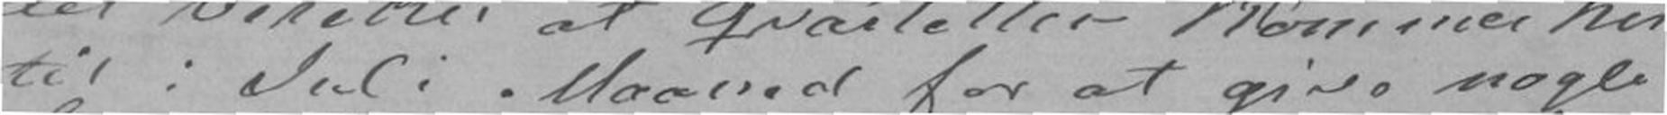til i Juli Maaned for at give nogle
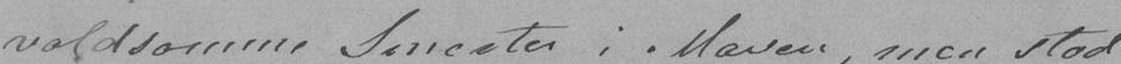voldsomme Smerter i Maven, men stod
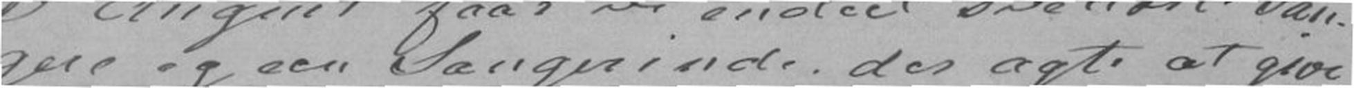gere og een Sangerinde der agte at give
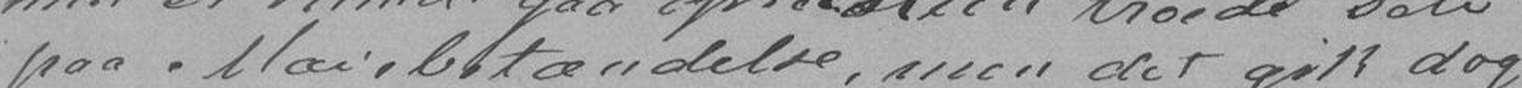paa Mavebetændelse, men det gik dog
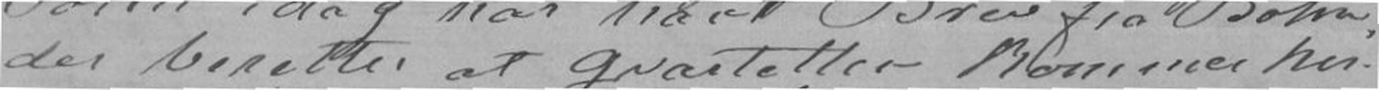der beretter at Qvartetten kommer her-
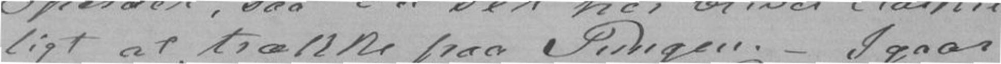ligt at trække paa Pungen. - Igaar
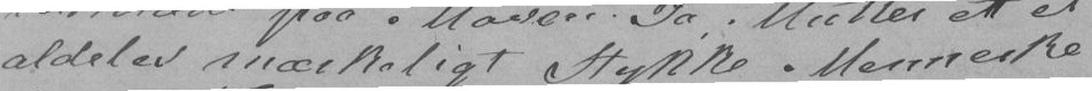aldeles mærkeligt Stykke Menneske
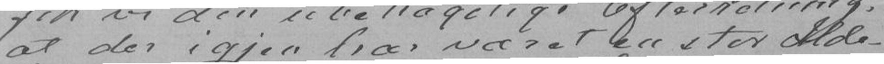at der igjen har været en stor Ilde-
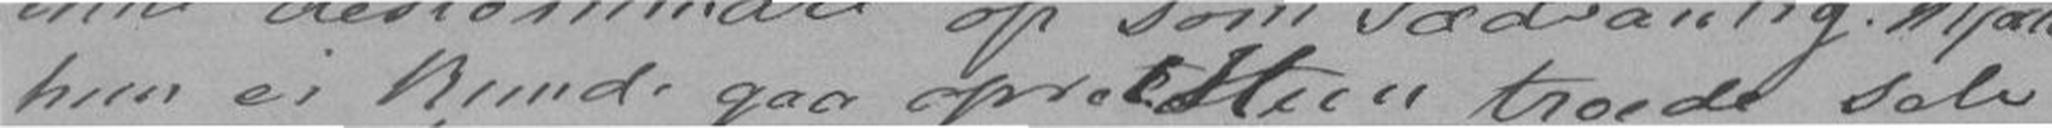hun ei kunde gaa opret. Hun troede selv
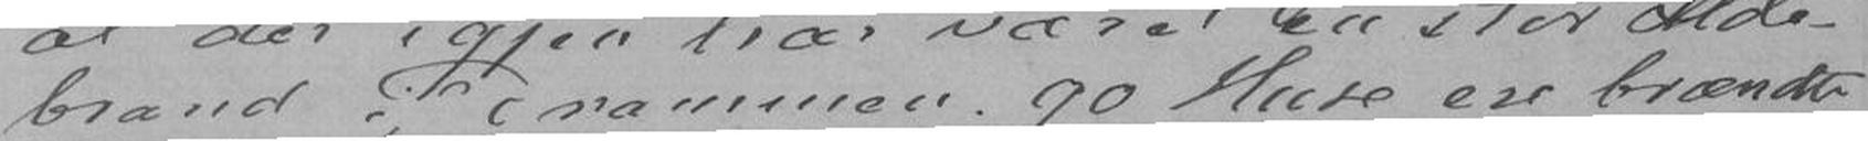brand i Drammen. 90 Huse ere brændte
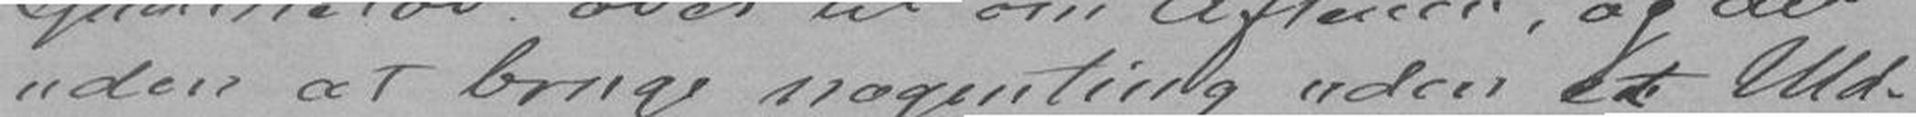uden at bruge nogenting uden et Uld-
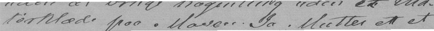tørklæde paa Mavven. Ja, Mutter er et
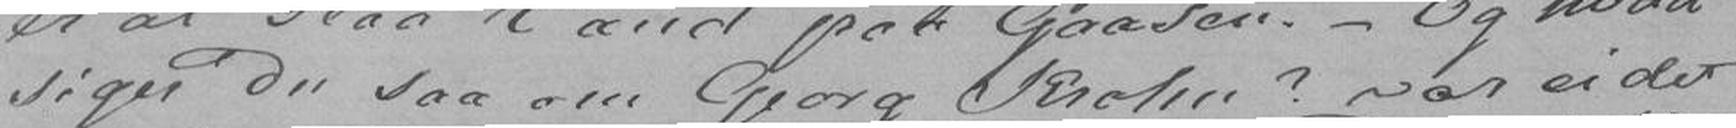siger Du saa om Georg Krohn? var ei det
